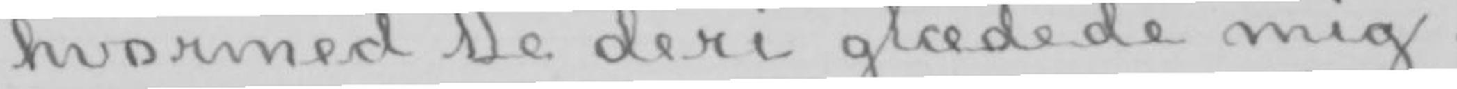hvormed De deri glædede mig.
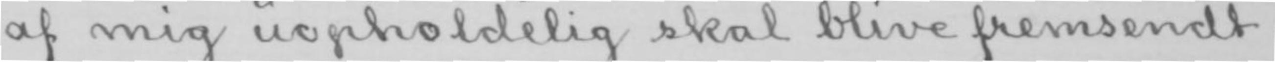af mig uopholdelig skal blive fremsendt.
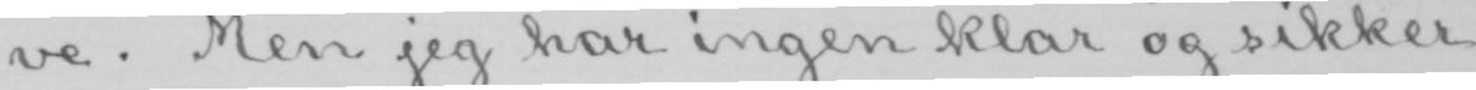ve. Men jeg har ingen klar og sikker
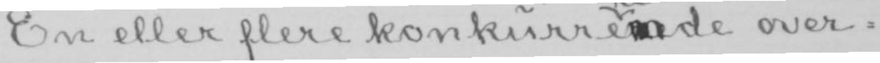En eller flere konkurrerende over-
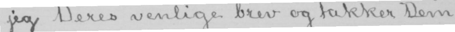jeg Deres venlige brev og takker Dem
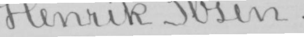Henrik Ibsen.
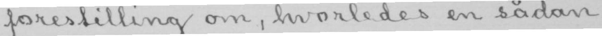forestilling om, hvorledes en sådan
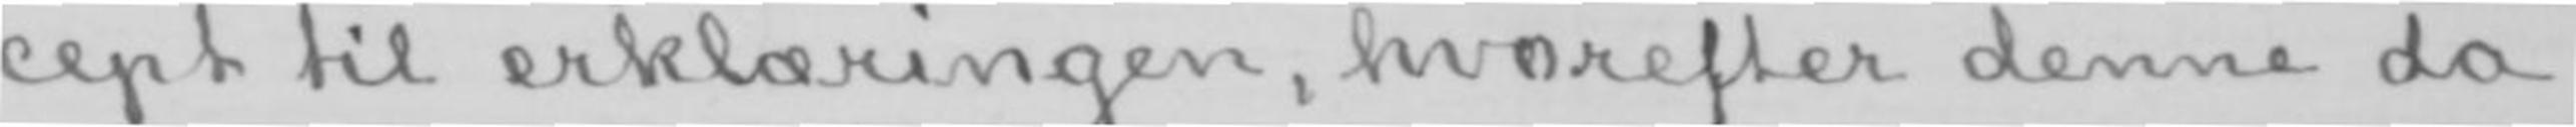cept til erklæringen, hvorefter denne da
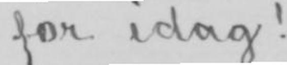for idag!
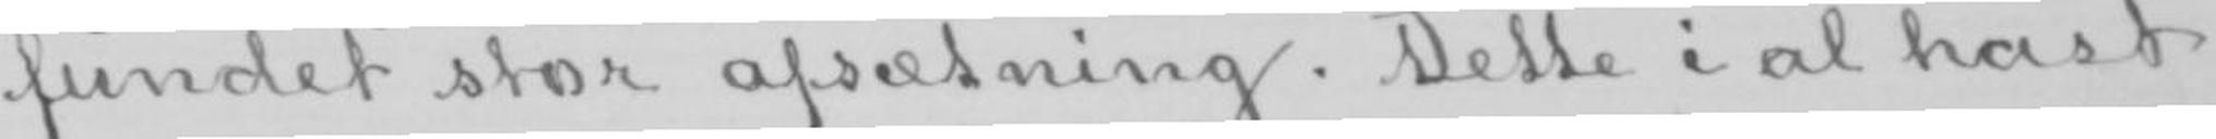fundet stor afsætning. Dette i al hast
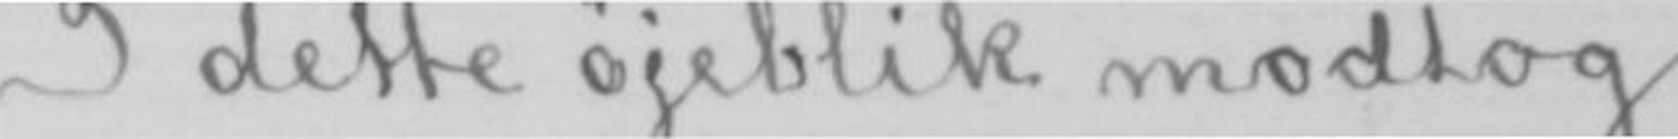I dette øjeblik modtog
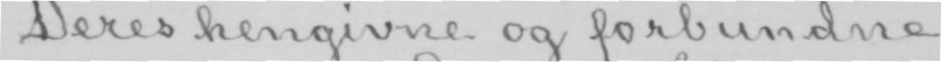Deres hengivne og forbundne
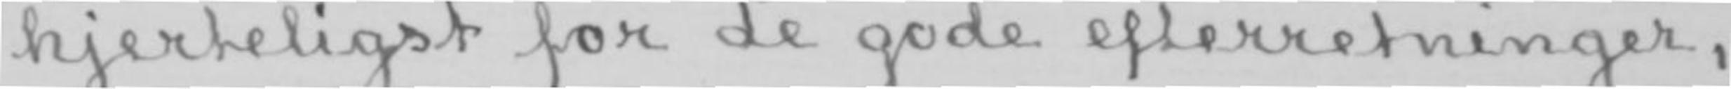hjerteligst for de gode efterretninger,
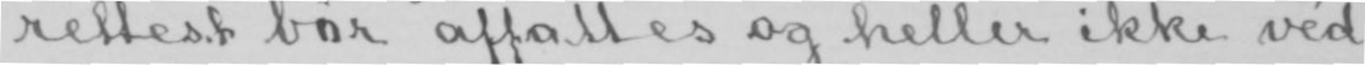rettest bør affattes og heller ikke véd
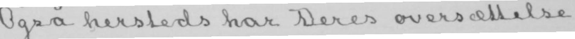Også hersteds har Deres oversættelse
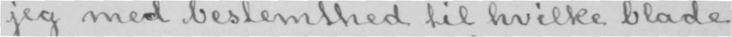jeg med bestemthed til hvilke blade
